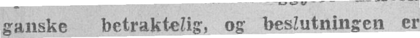ganske betraktelig, og beslutningen er
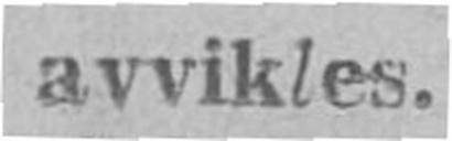avvikles.
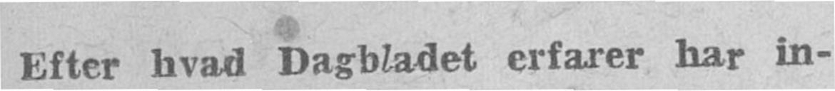Efter hvad Dagbladet erfarer har in-
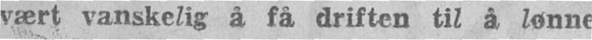vært vanskelig å få driften til å lønne
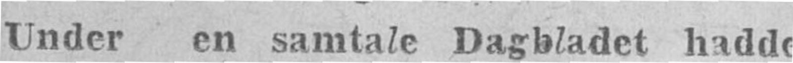Under en samtale Dagbladet hadde
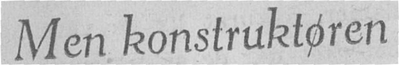Men konstruktøren
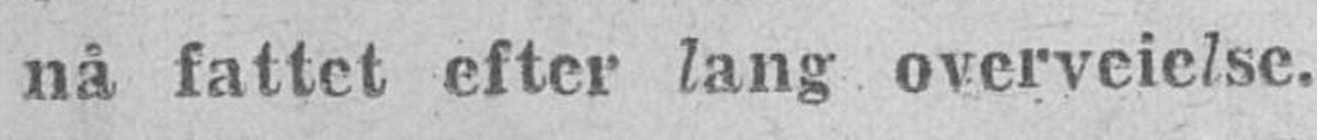nå fattet efter lang overveielse.
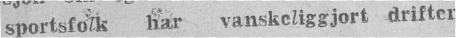sportsfolk har vanskeliggjort driften
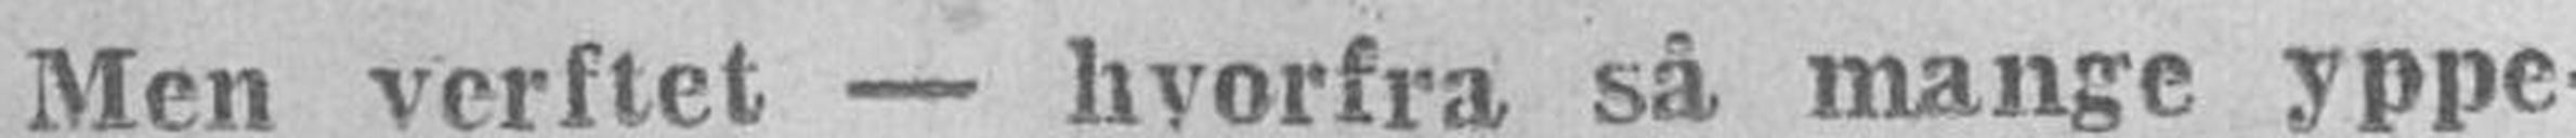Men verftet — hvorfra så mange yppe-
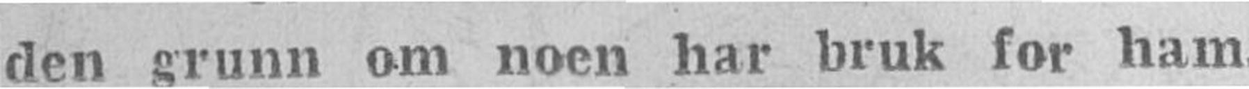den grunn om noen har bruk for ham.
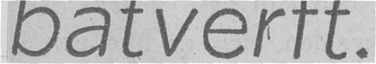båtverft.
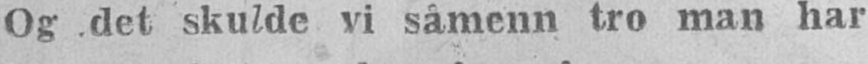Og det skulde vi såmenn tro man har!
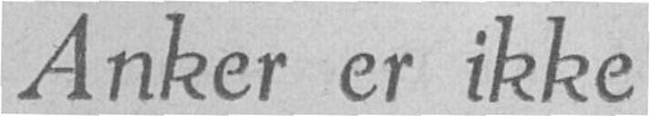Anker er ikke
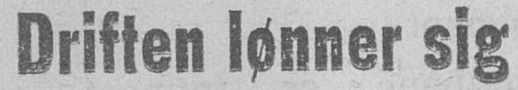Driften lønner sig
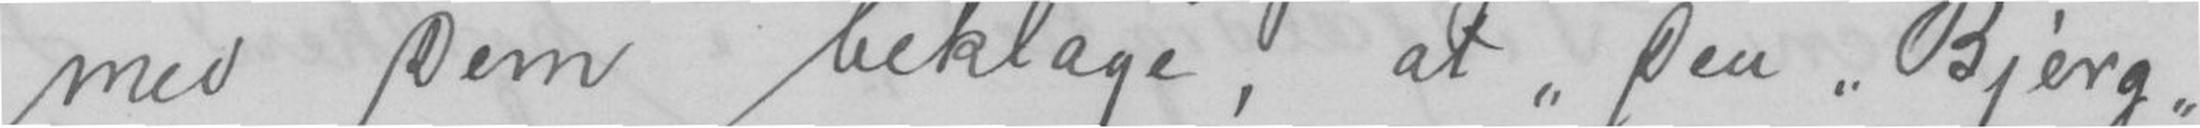med Dem beklage, at "Den Bjerg-
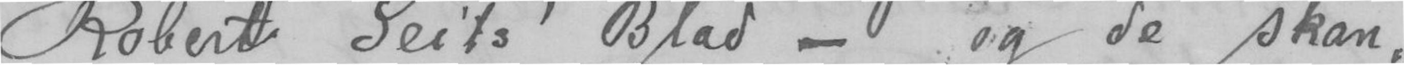Robert Seits' Blad - og de skan
